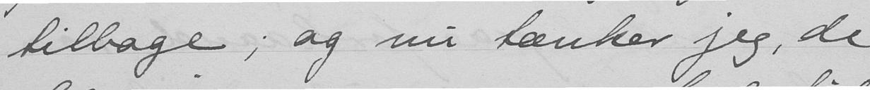tilbage; og nu tænker jeg, de
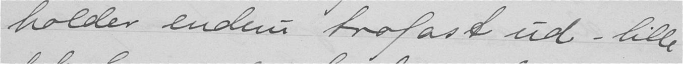holder endnu trofast ud - lille
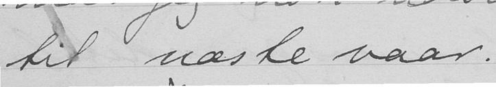til næste vaar.
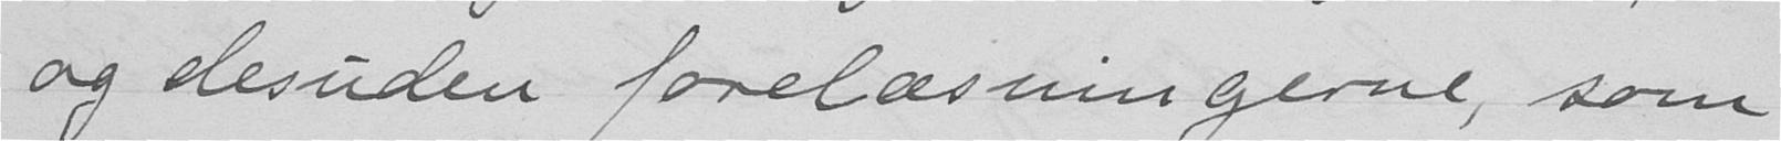og desuden forelæsningerne, som
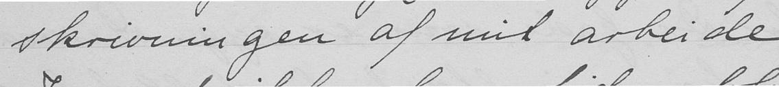skrivningen af mit arbeide.
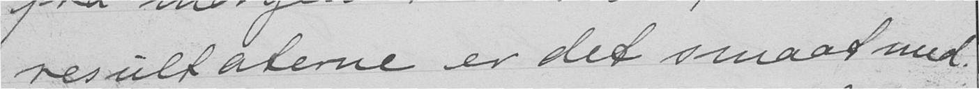resultaterne er det smaat med.
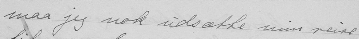maa jeg nok udsætte min reise
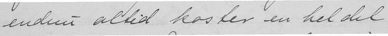endnu altid koster en hel del
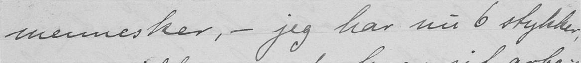mennesker, - jeg har nu 6 stykker,
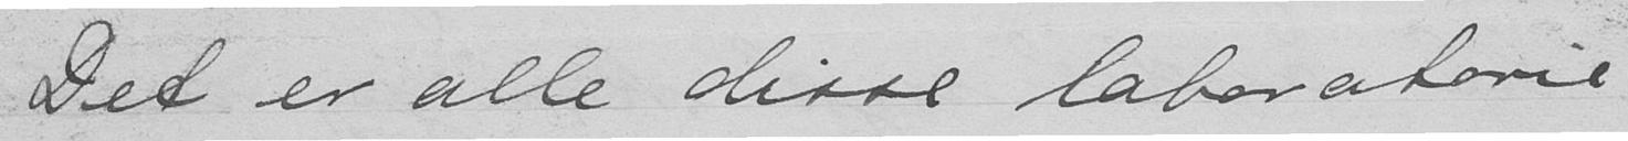Det er alle disse laboratorie-
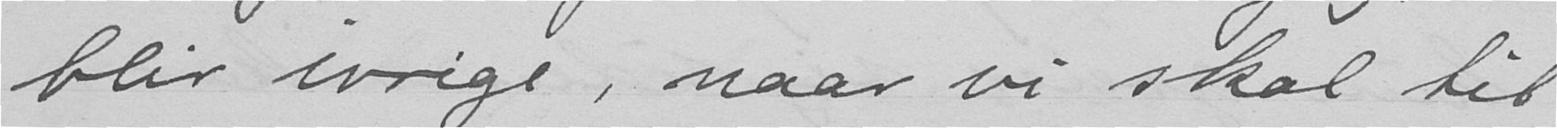blir ivrige, naar vi skal til
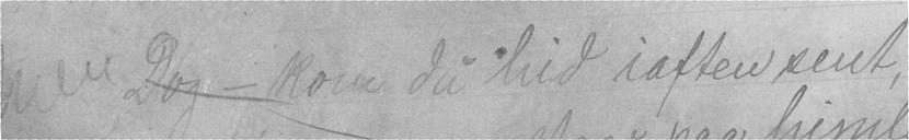Dog - kom on hid iaften sent,
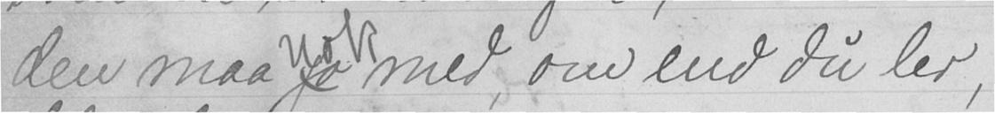den maa nok med, om end du ler,
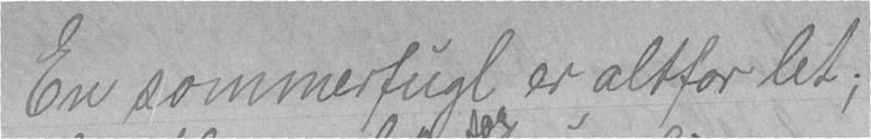En sommerfugl er altfor let;
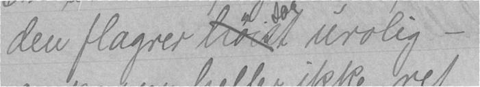den flagrer saa urolig -
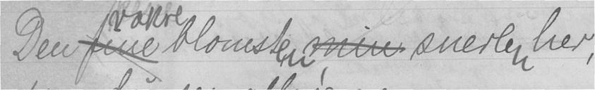Den vakre blomsten min snerlen her,
